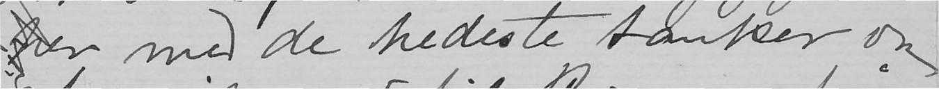her med de hedeste tanker om
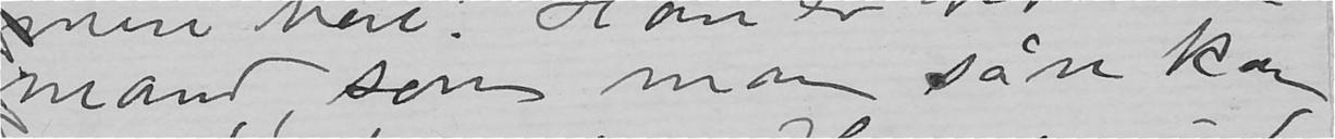mand, som man sån kan
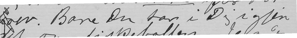brev. Bare Du tar i Dig igjen
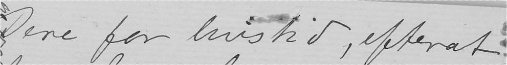Dere for livstid, efterat
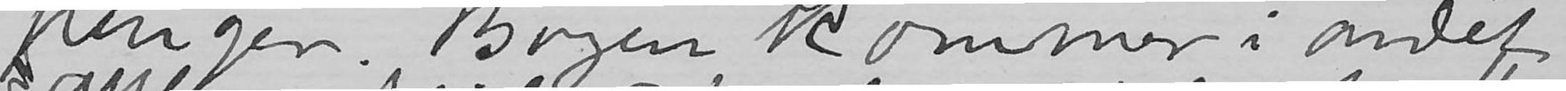penger. Bogen kommer i andet
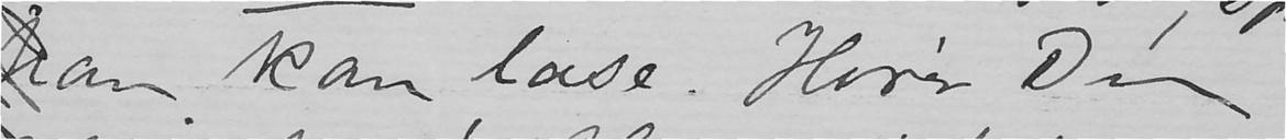han kan læse. Hører Du
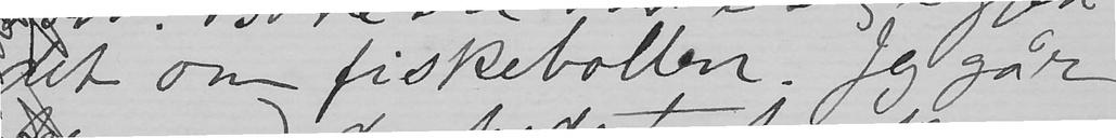det om fiskebollen. Jeg går
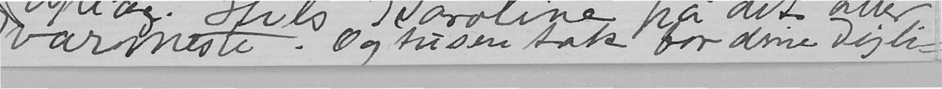varmeste. Og tusen tak for dine dejli-
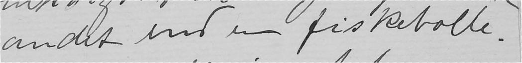andet end en fiskebolle.
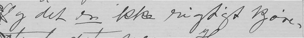og det er ikke rigtigt kjære-
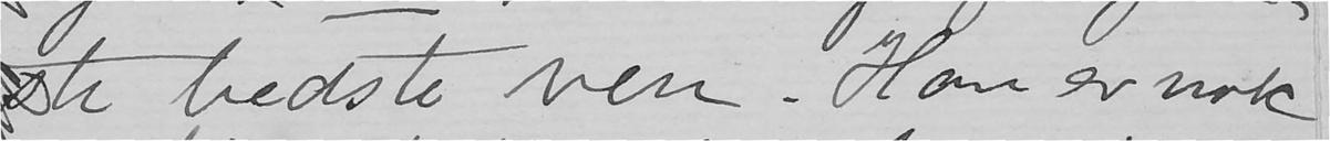ste bedste ven. Han er nok
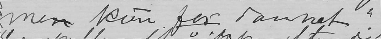men kun for dannet!
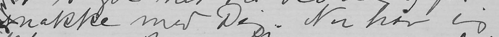snakke med Dig. Nu har jeg
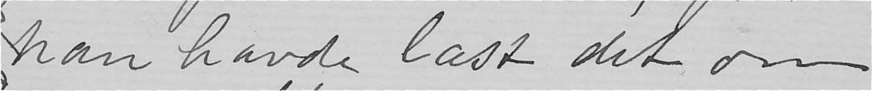han havde læst det om
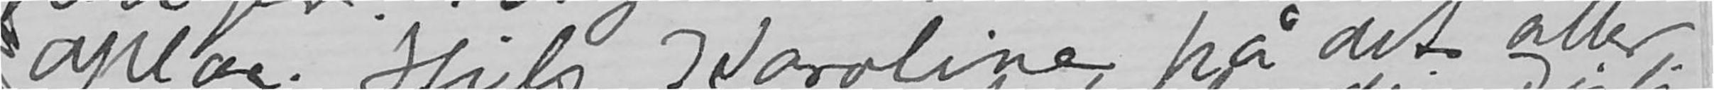oplag. Hils Karoline på det aller
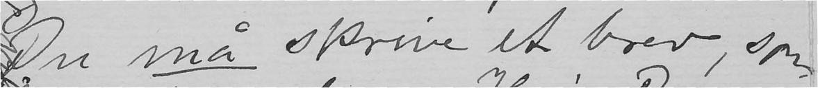Du må skrive et brev, som
